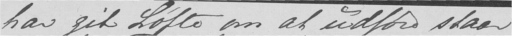har git Løfte om at udføre staar
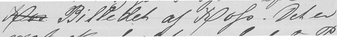Ras Billedet af Rofs. Det er
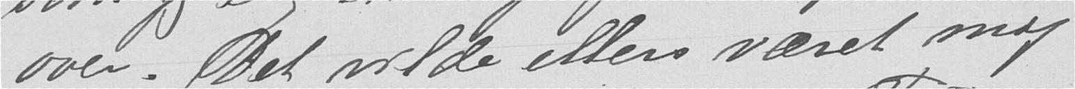over. Det vilde ellers været mig
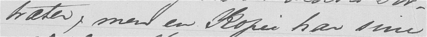træter, men en Kopie har sine
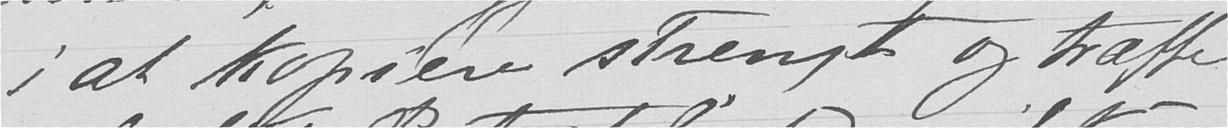i at kopiere strengt og træffe
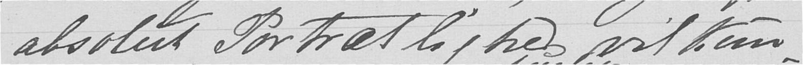absolut Portrætlighed, vil kun-
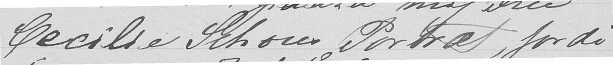Cecilie Schous Portræt, fordi
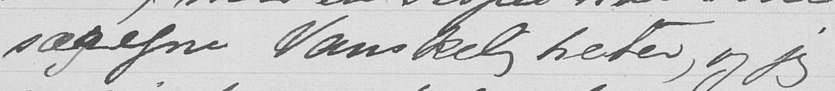særegne Vanskeligheter, og jeg
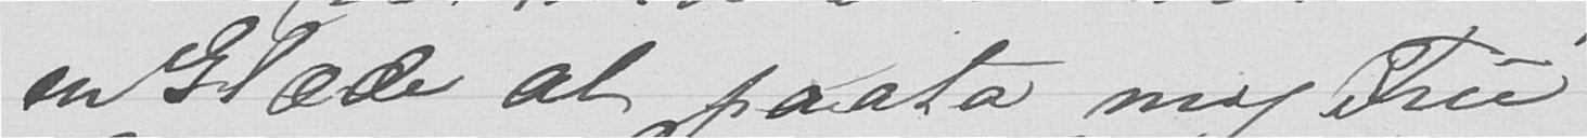en Glæde at paata mig Fru
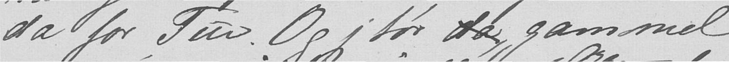da for Tur. Og jeg tør da, gammel
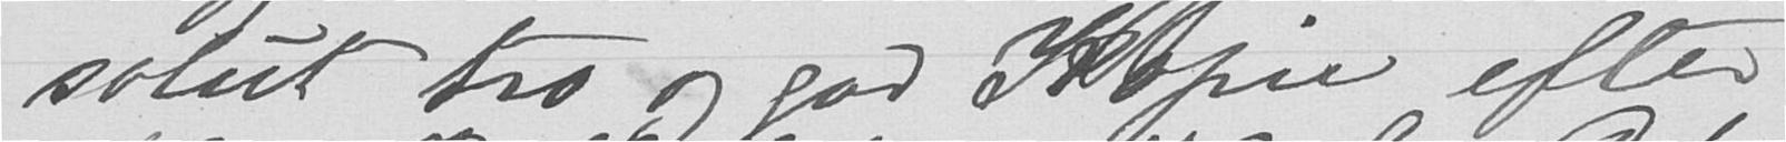solut tro og god Kopie efter
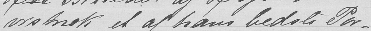vistnok et af hans bedste Por-
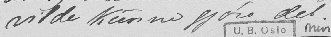vilde kunne gjøre det
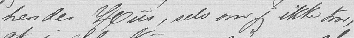hendes Hus, selv om jeg ikke tror,
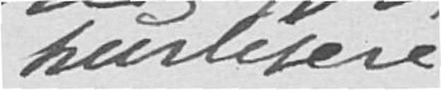hurtigere
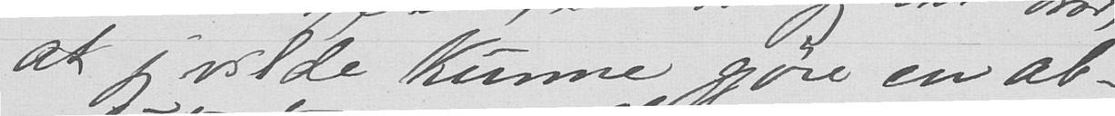at jeg vilde kunne gjøre en ab-
